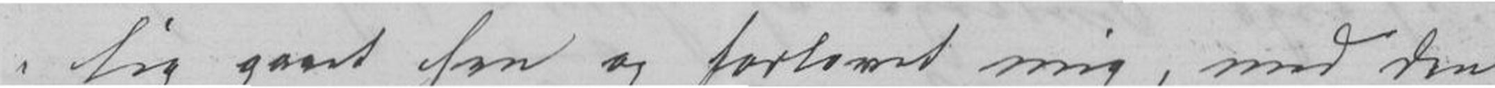-lig gaaet hen og forlovet mig, med den
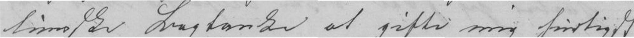lumske bagtanke at gifte mig, hurtigst
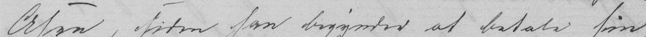Asen siden han begynder at betale sin
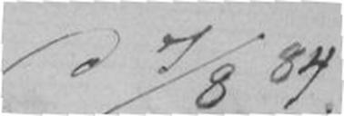d 7/8 84.
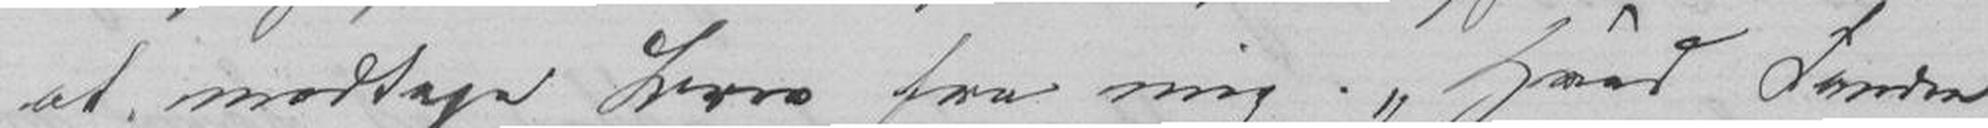at modtage brev fra mig. Hvad fanden
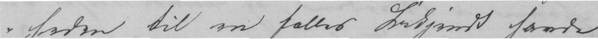heden til en halvt Bekjendt havde
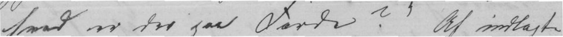hvad er der paa Færde ? Af indlagte
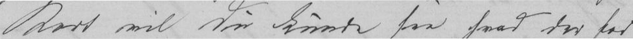kort vil Du kunde see hvad der for
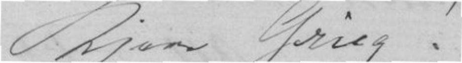Kjære Grieg!
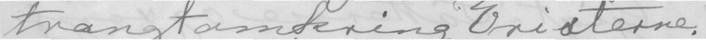trangt omkring Vristerne,
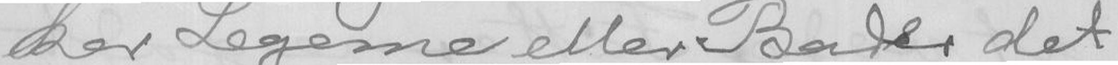ker Legeme eller Bader det
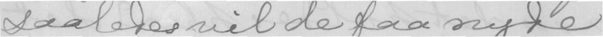saaledes vil de faa nyde
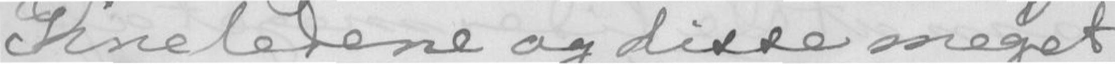Kneledene og disse meget
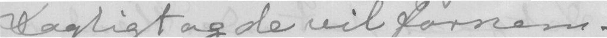Dagligt og de vil fornem-
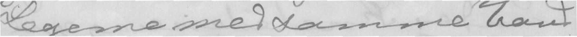Legememed samme Vand
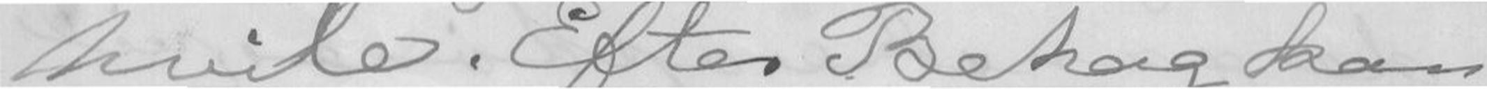hvile. Efter Behag kan
